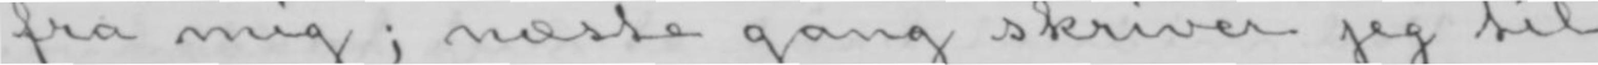fra mig; næste gang skriver jeg til
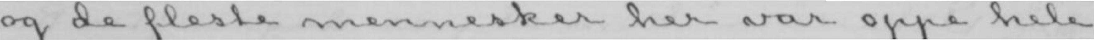og de fleste mennesker her var oppe hele
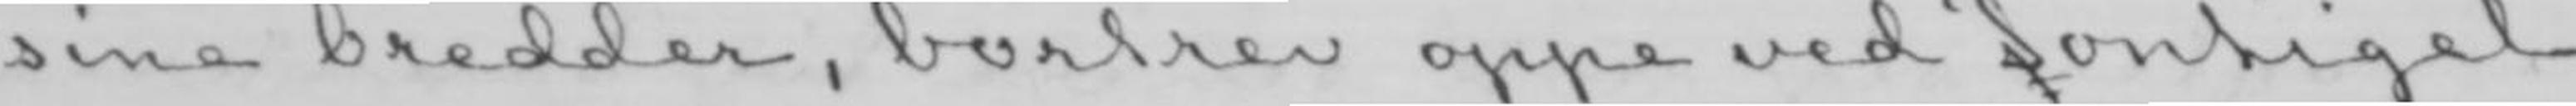sine bredder, bortrev oppe ved Pontigel
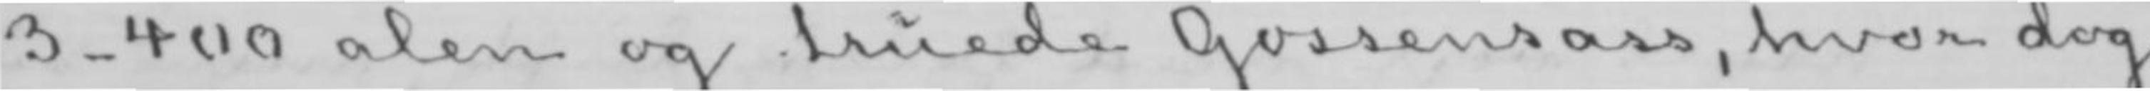3-400 alen og truede Gossensass, hvor dog
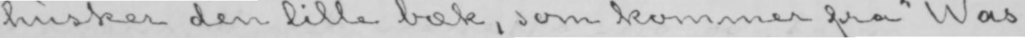husker den lille bæk, som kommer fra «Was-
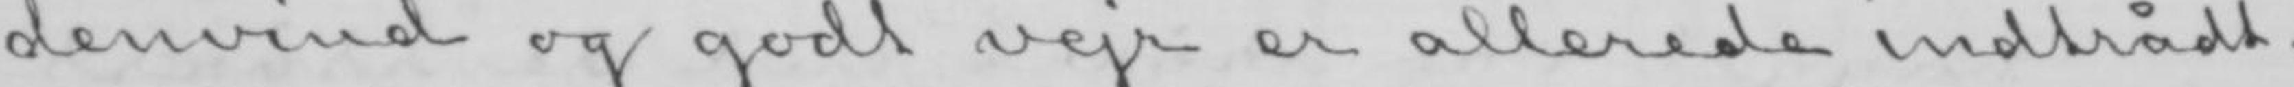denvind og godt vejr er allerede indtrådt.
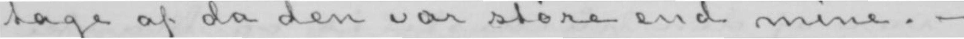tage af da den var støre end mine.-
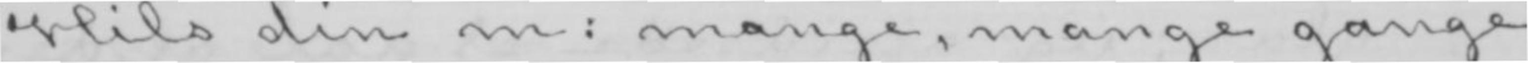Hils din m: mange, mange gange
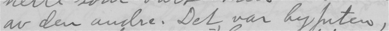av den andre. Det var byfuten,
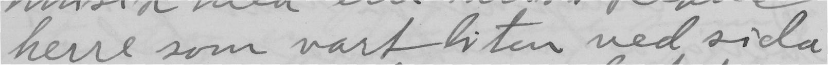herre som vart liten ved sida
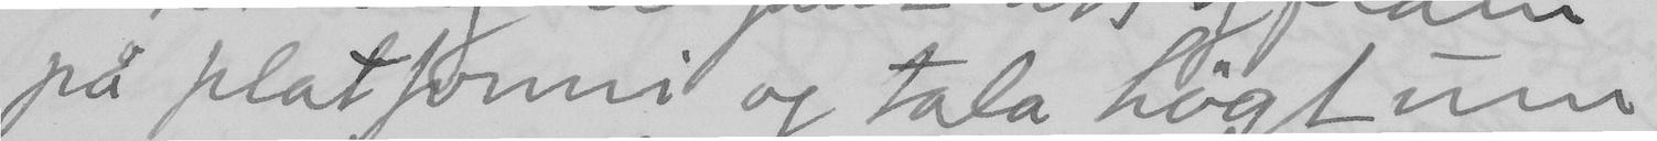på platformi og tala högt um
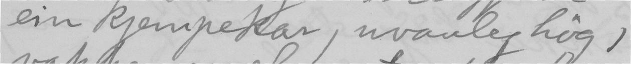ein kjempekar, uvanleg hög,
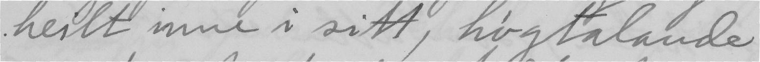heilt inne i sitt, högtalande
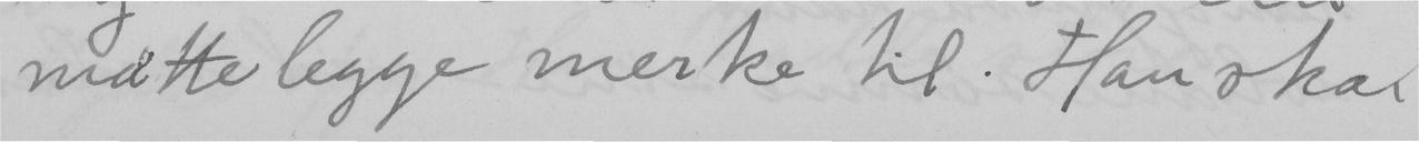måtte legge merke til. Han skal
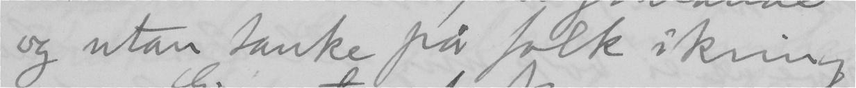og utan tanke på folk ikring
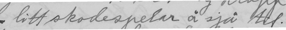litt skodespelar å sjå til.
